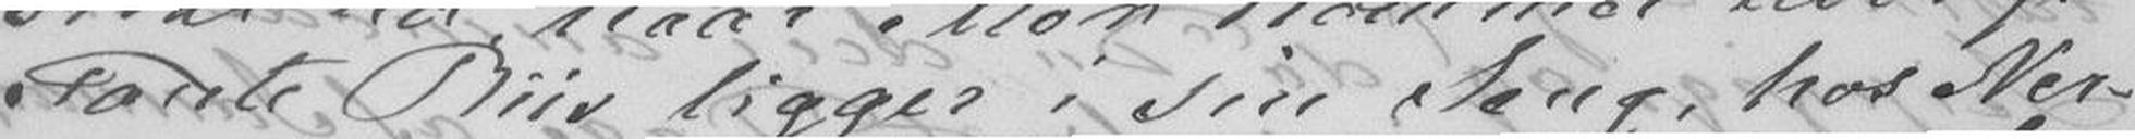Tante Riis ligger i sin Seng, hos Ner-
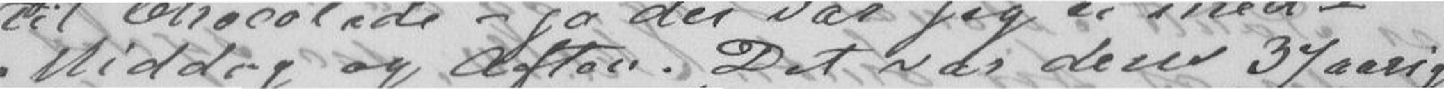Middag og Aften. Det var deres 37 aarige
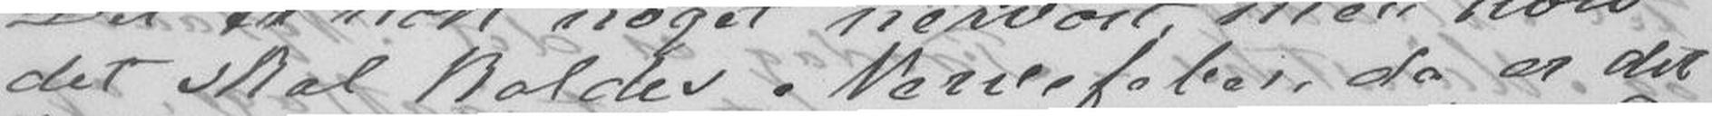det skal koldes Nervefeber, da er det
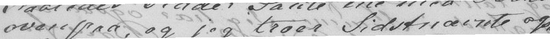ovenpaa, og jeg troer Sidstnævnte ogsaa
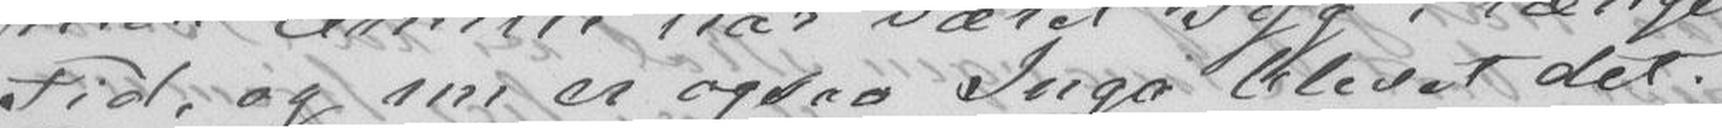Tid, og nu er ogsaa Inga blevet det.
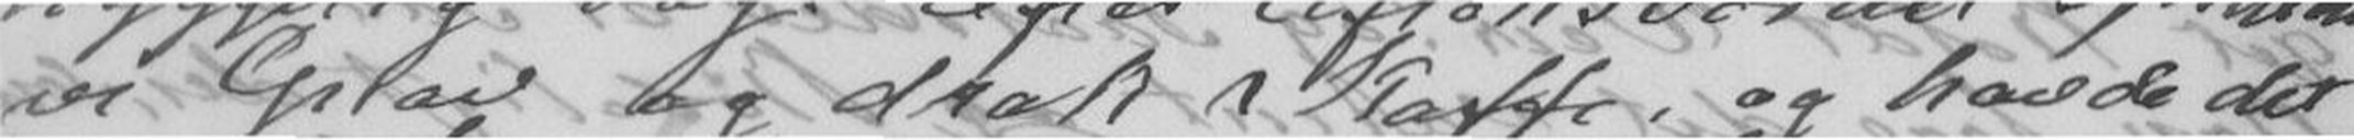vi Gnav og drak Kaffe, og havde det
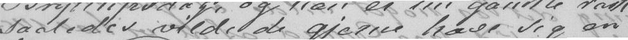saaledes vilde de gjerne have sig en
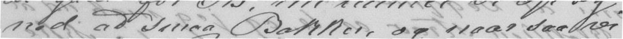ned ad smaa Bakker, og naar saa vi
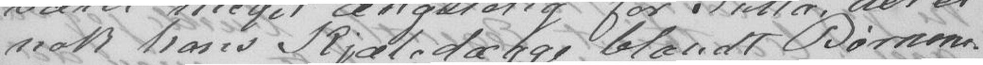nok hans Kjæledægge blandt Børnene.
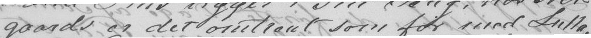gaards er det omtrænt som før med Lulla,
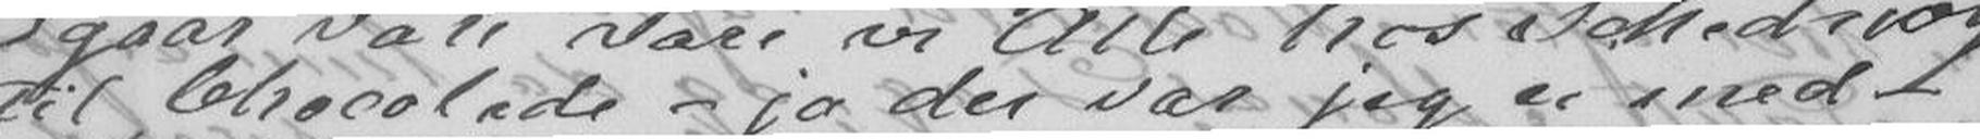til Chocolade - jo der var jeg ei med -
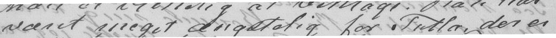været meget ængstelig for Tulla, der er
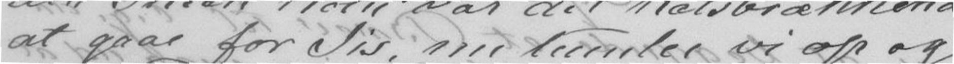at gaae for Iis, nu tumler vi op og
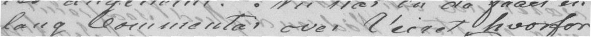lang Commentar over Veiret hvorfor
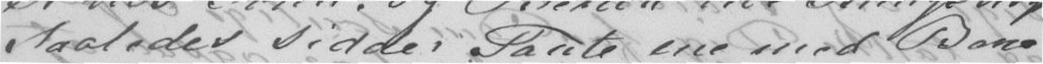Saaledes sidde Tante ene med Bene
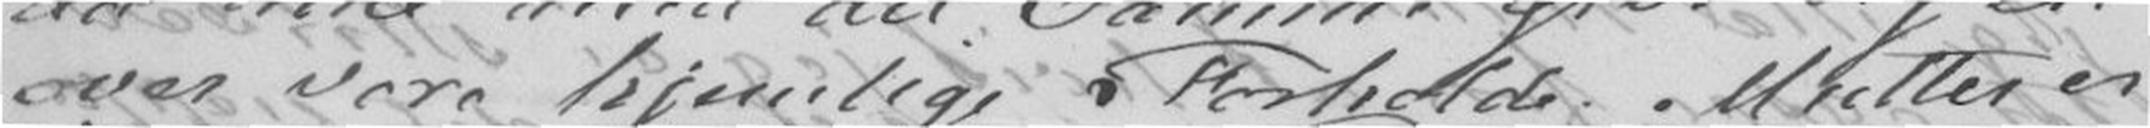over vore hjemlige Forholde. Mutter er
